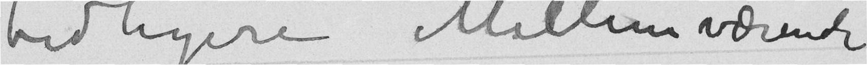tidligere Mellemværende
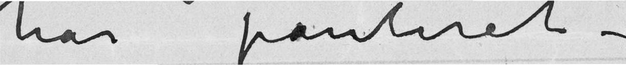har panteret –
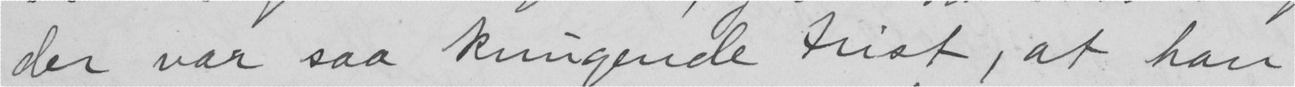der var saa knugende trist, at han
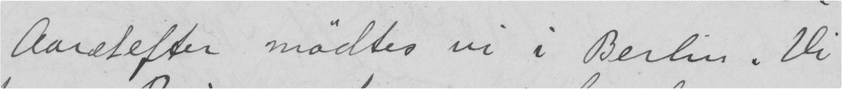Aaret efter mødtes vi i Berlin. Vi
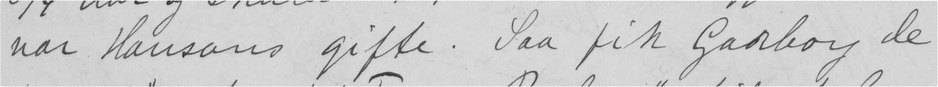var Hansons gifte. Saa fik Garborg de
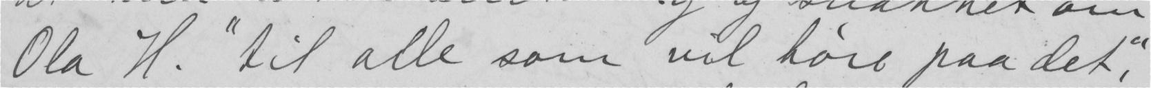Ola H. "til alle som vil høre paa det."
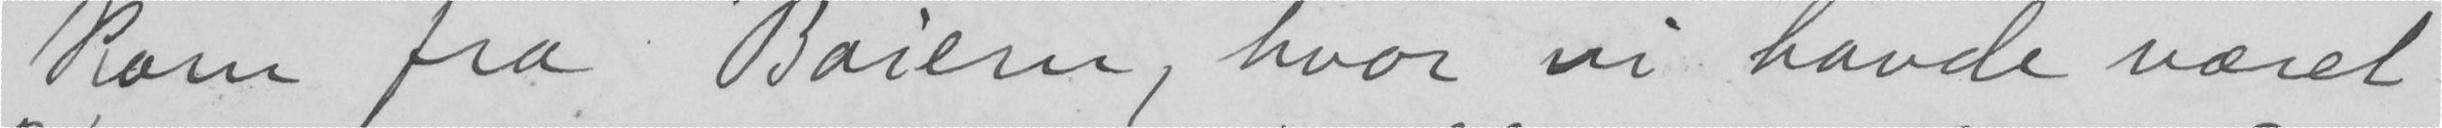kom fra Baiern, hvor vi havde været
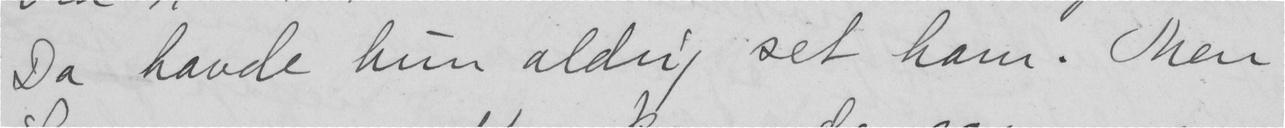Da havde hun aldrig set ham. Men
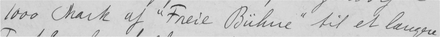1000 Mark af "Freie Bühne" til et længere
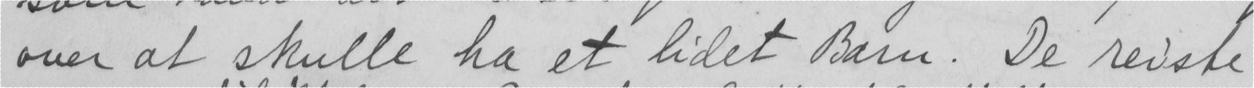over at skulle ha et lidet Barn. De reiste
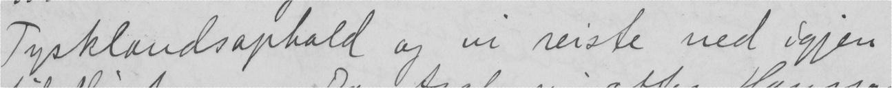Tysklandsophold og vi reiste ned igjen
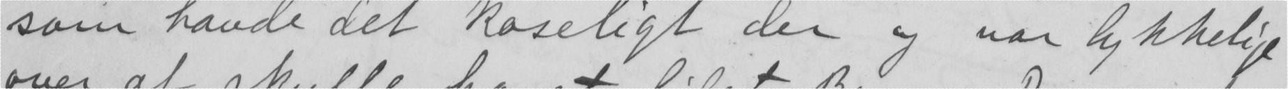som havde det koseligt der og var lykkelige
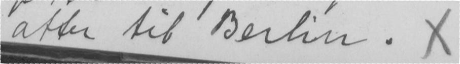atter til Berlin. x
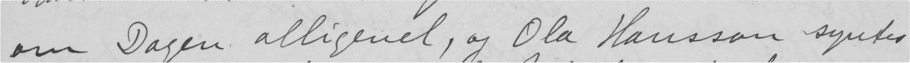om Dagen alligevel, og Ola Hansson syntes
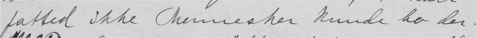fatted ikke Mennesker kunde bo der.
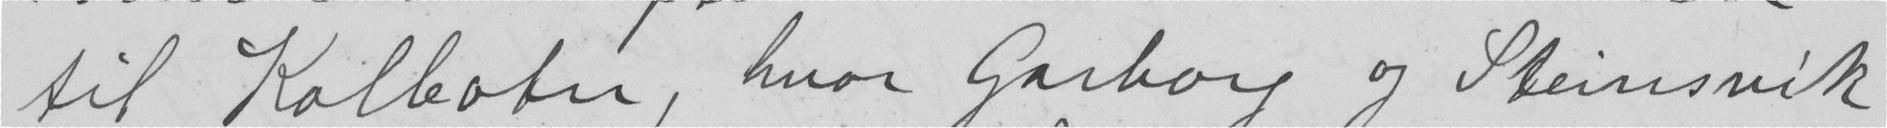til Kolbotn, hvor Garborg og Steinsvik
